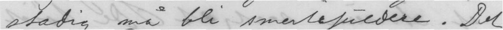stadig må bli smertefuldere. Det
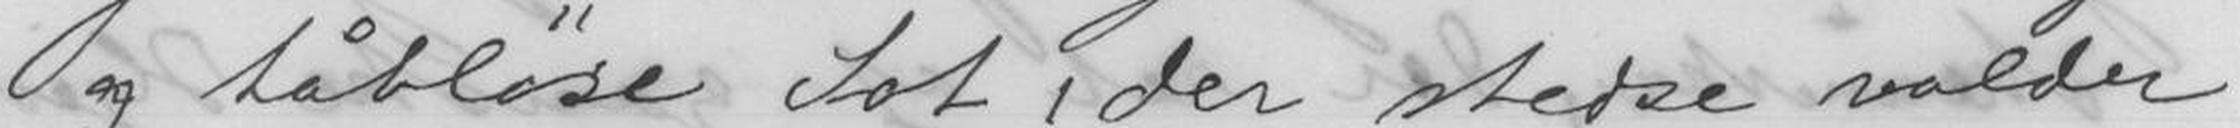og håbløse Sot, der stedse volder
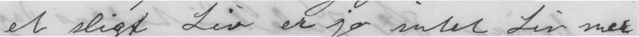et sligt Liv er jo intet Liv mer.
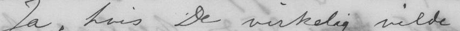Ja, hvis De virkelig vilde
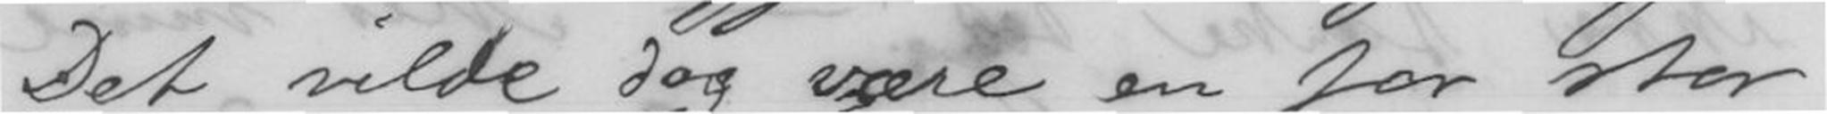Det vilde dog være en for stor
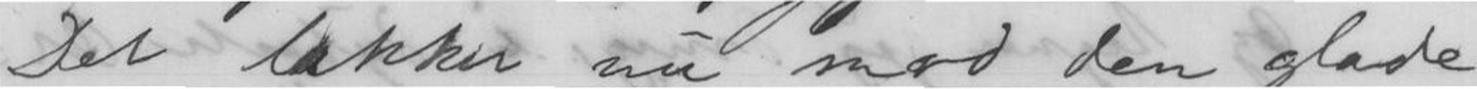Det lakker nu mod den glade
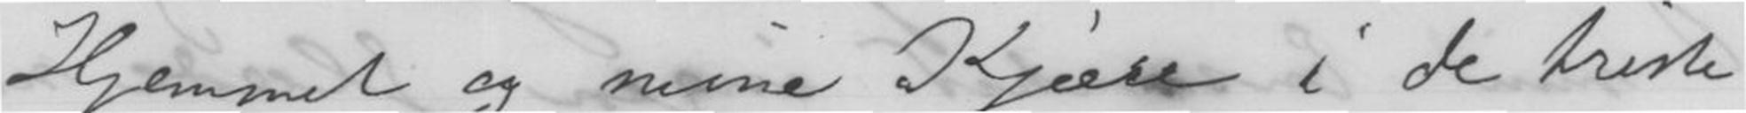Hjemmet og mine Kjære i de triste
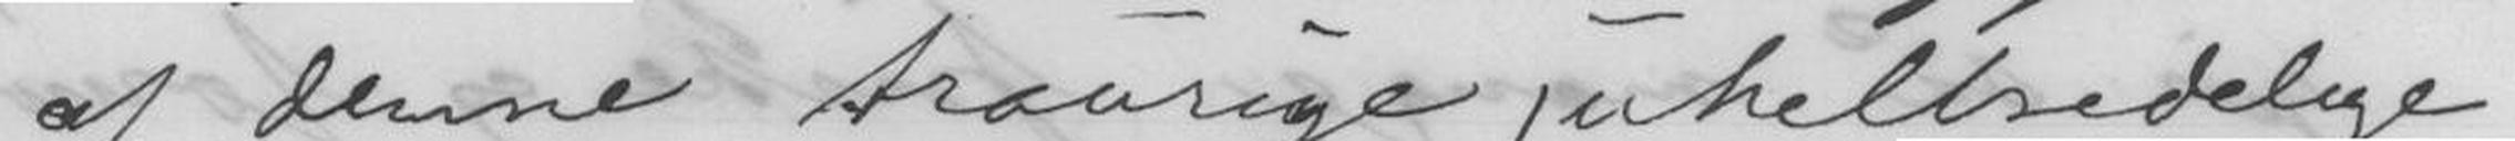af denne traurige, uhelbredelige
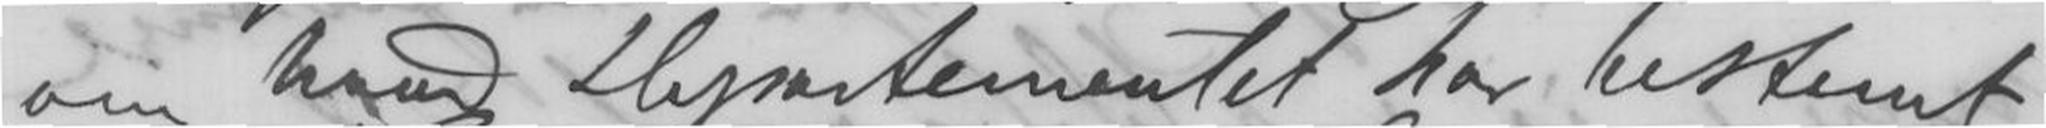om hvad Departementet har bestemt
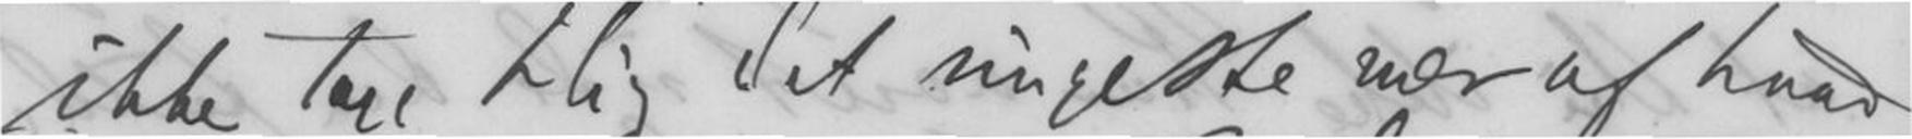ikke tage Dig det ringeste nær af hvad
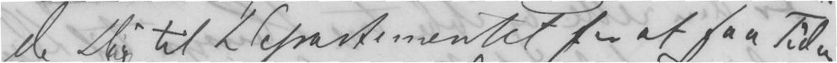de Dig til Departementet for at faa Tiden
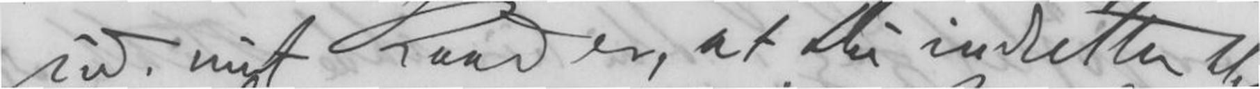ud; mit Raad er, at Du indretter Dig
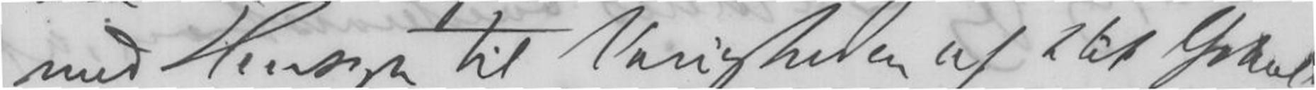med Hensyn til Varigheden af Dit Ophold
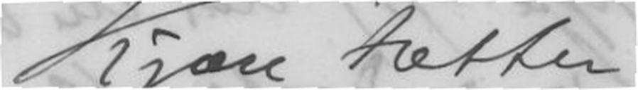Kjære Fætter
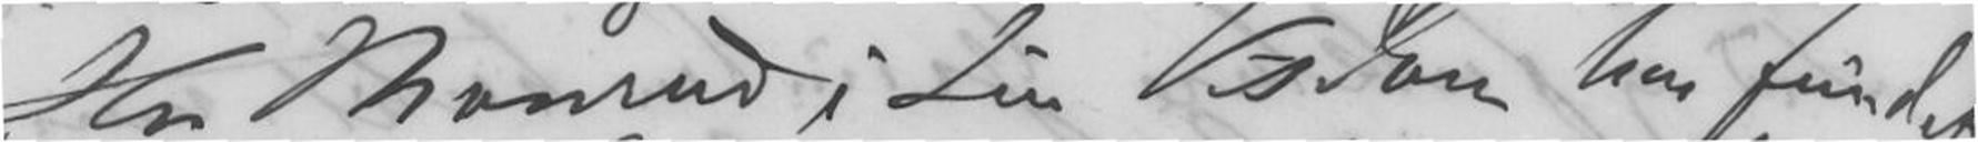Hrr Monrad i sin Visdom har fundet
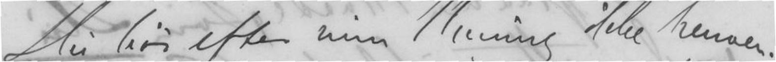Du bør efter min Mening ikke henven-
